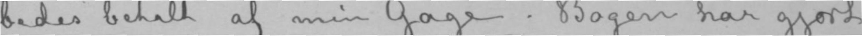bedes betalt af min Gage. Bogen har gjort
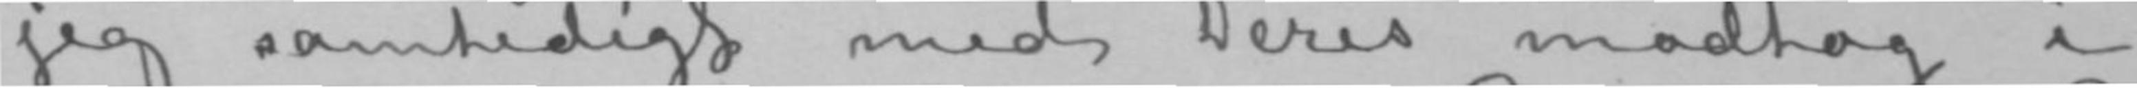jeg samtidigt med Deres modtog i
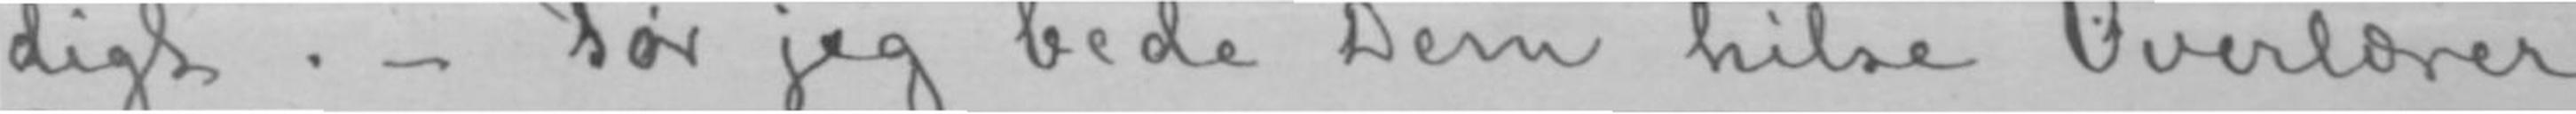digt.- Tør jeg bede Dem hilse Overlærer
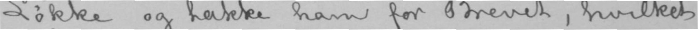Løkke og takke ham for Brevet, hvilket
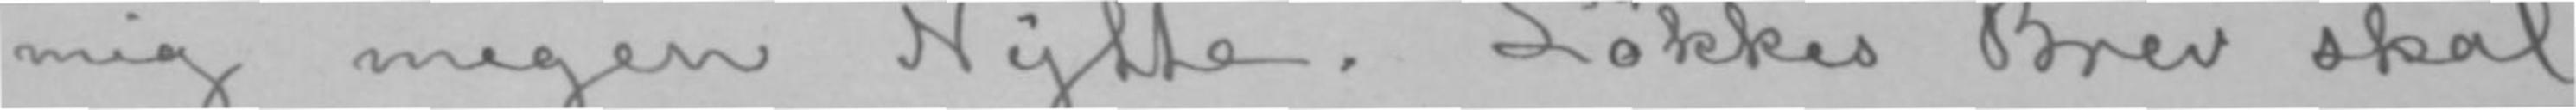mig megen Nytte. Løkkes Brev skal
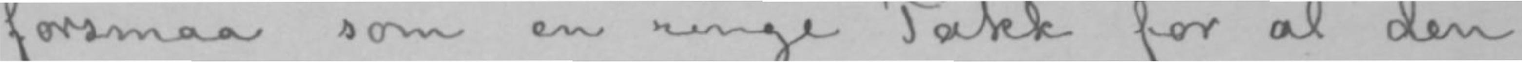forsmaa som en ringe Takk for al den
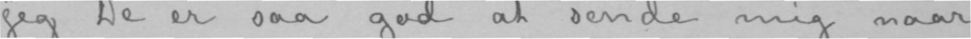jeg De er saa god at sende mig naar
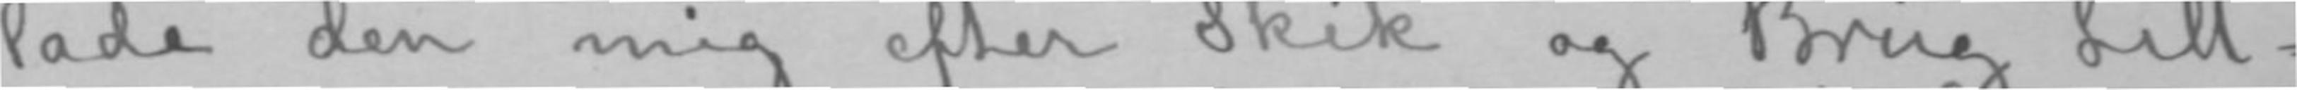lade den mig efter Skik og Brug till-
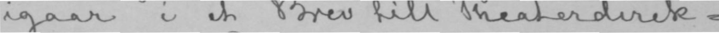jeg igaar i et Brev till Theaterdirek-
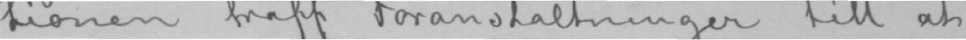tionen traff Foranstaltninger till at
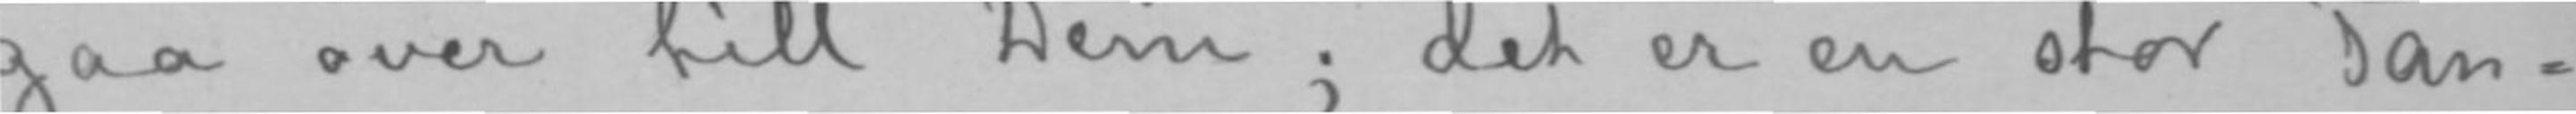gaa over till Dem; det er en stor Tan-
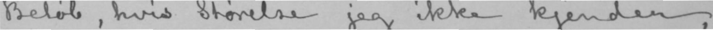Beløb, hvis Størelse jeg ikke kjender,
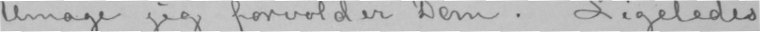Umage jeg forvolder Dem. Ligeledes
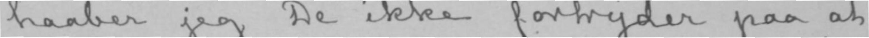haaber jeg De ikke fortryder paa at
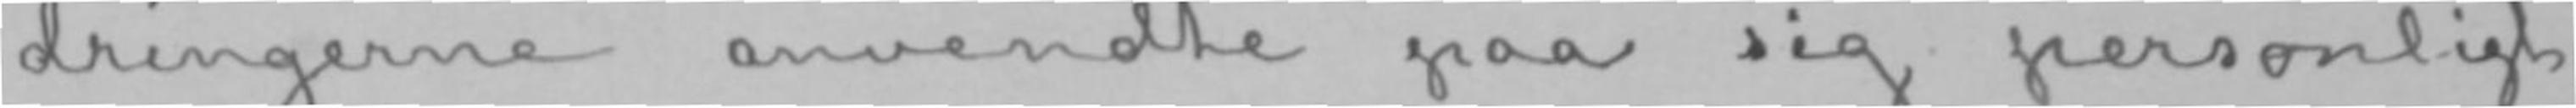dringerne anvendte paa sig personligt,
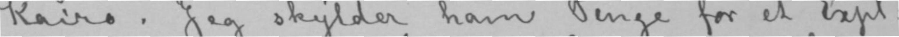Kairo. Jeg skylder ham Penge for et Expl:
